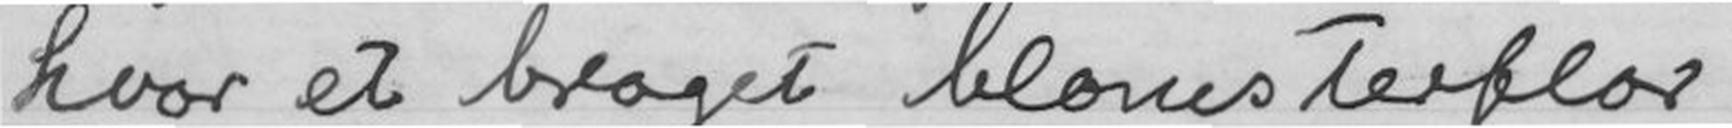hvor et broget blomsterflor
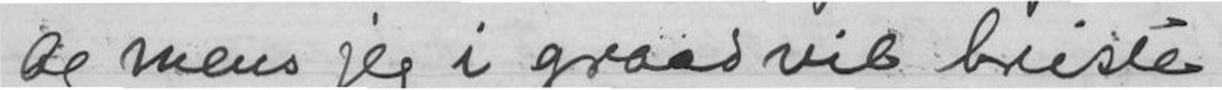Og mens jeg i graad vil briste
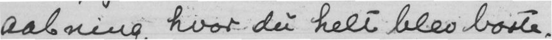Aabning, hvor du helt blev borte.
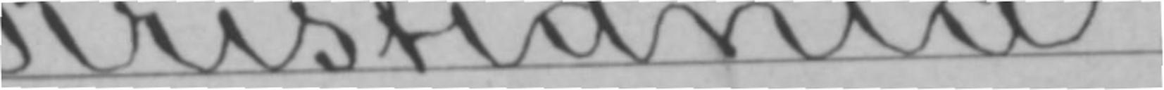Kristiania.
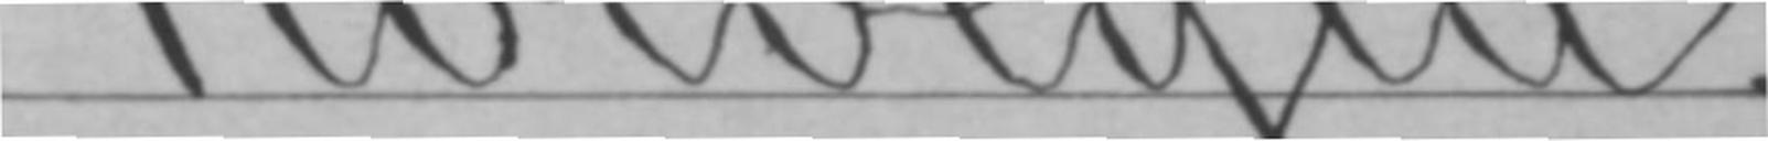Norvegia.
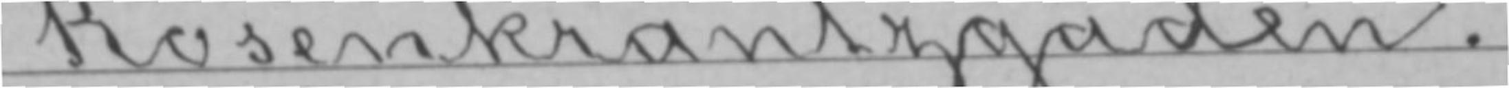Rosenkrantzgaden.
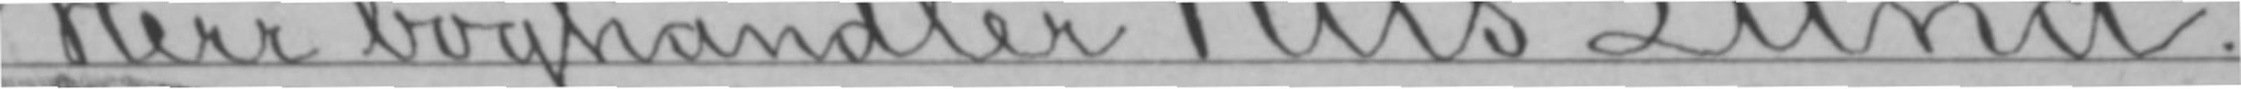Herr boghandler Nils Lund.
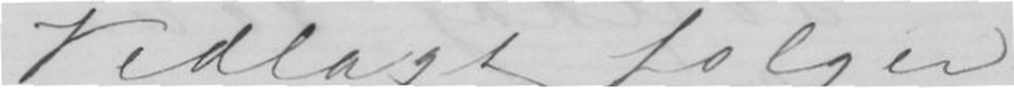Vedlagt følger
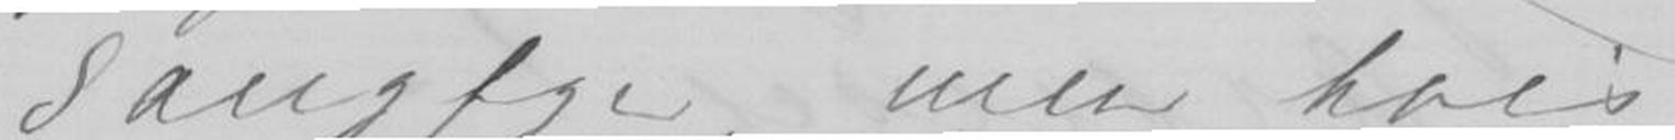sangfgen, men hvis
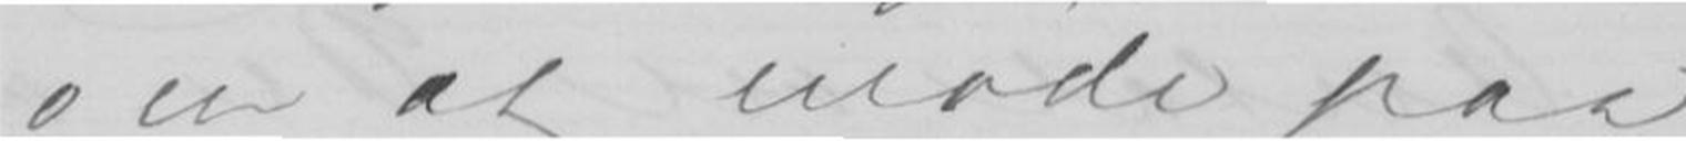om at møde paa
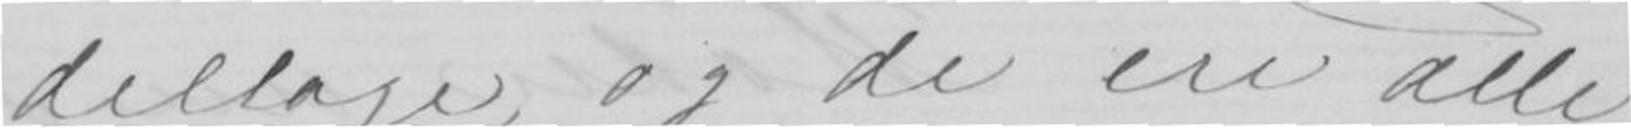deltage, og de ere alle
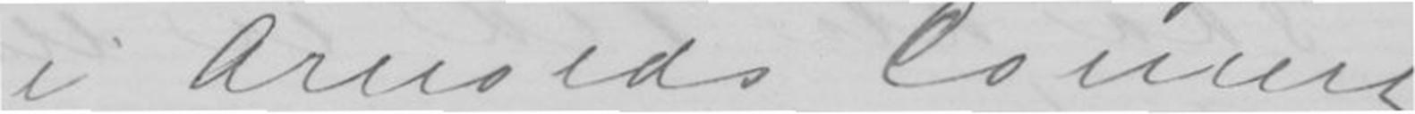i Arnolds Concert.
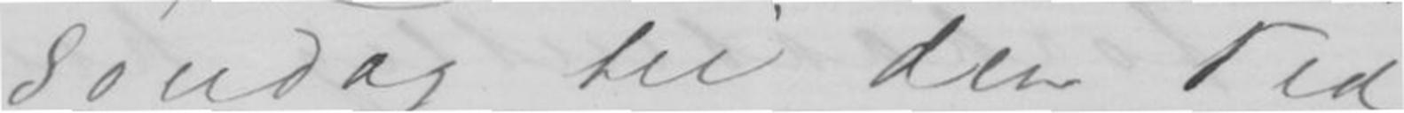Søndag til den Tid
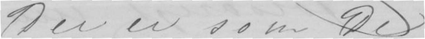Der er som De
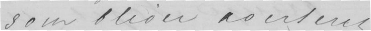som blive averteret
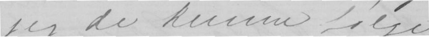jeg de kunne følge
